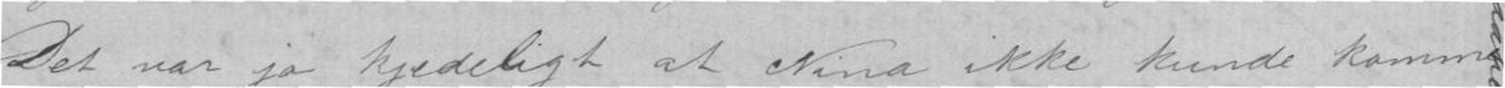Det var jo kjedeligt at Nina ikke kunde komme
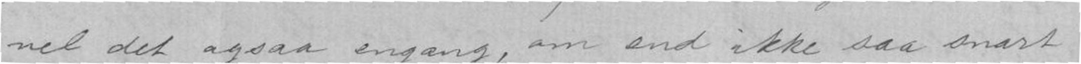vel det ogsaa engang, om end ikke saa snart
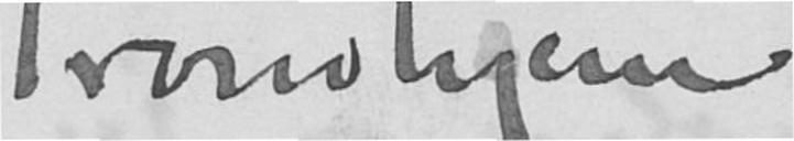Trondhjem
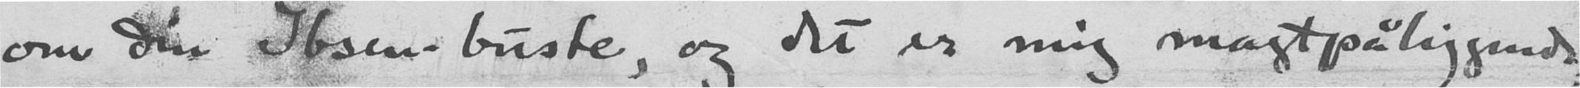om din Ibsen-buste, og det er mig magtpåliggende
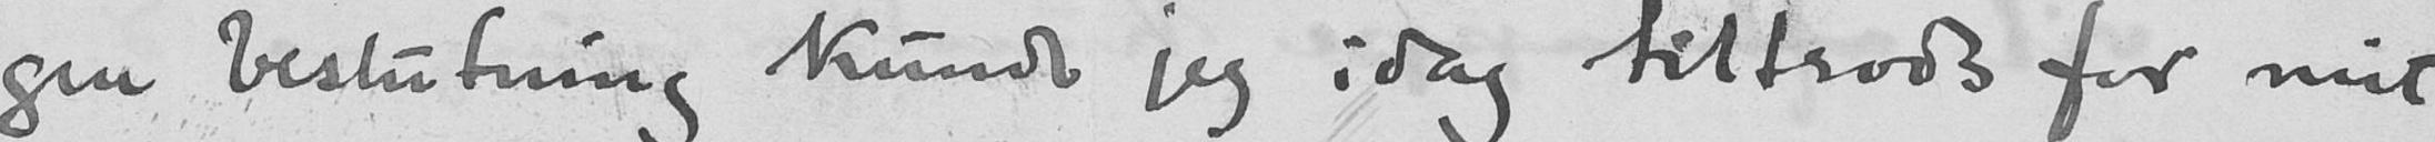gen beslutning kunde jeg idag tiltrods for mit
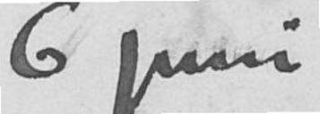6 juni
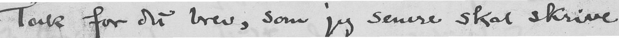Tak for dit brev, som jeg senere skal skrive
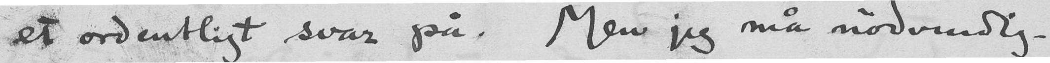et ordentligt svar på. Men jeg må nødvendig-
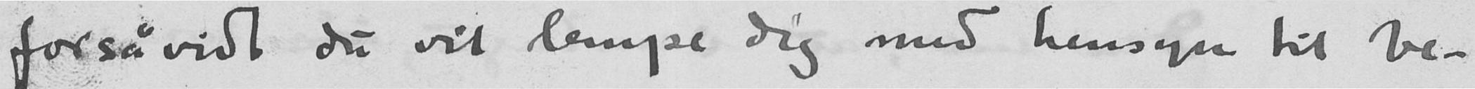forsåvidt du vil lempe dig med hensyn til be-
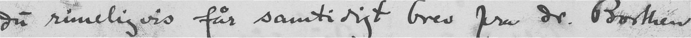du rimeligvis får samtidigt brev fra dr. Borthen
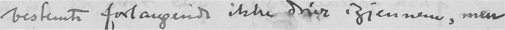bestemte forlangende ikke drive igjennem, men
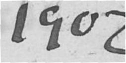1902
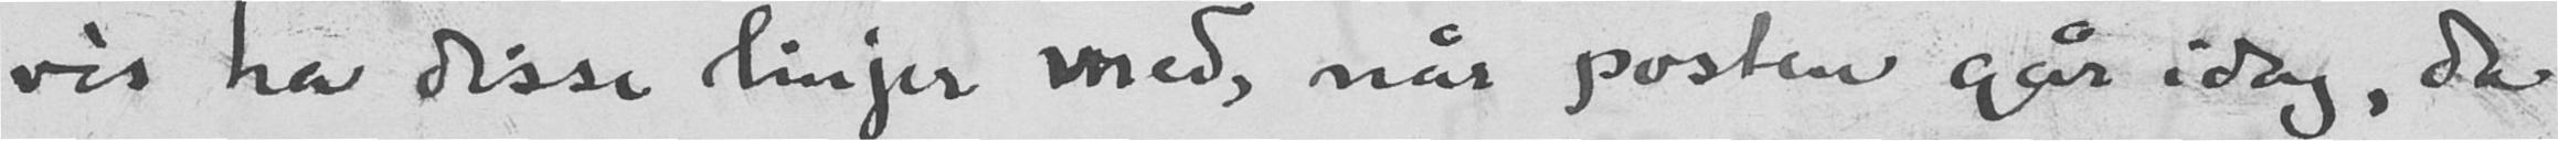vis ha disse linjer med, når posten går idag, da
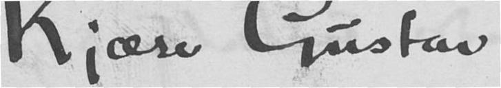Kjære Gustav
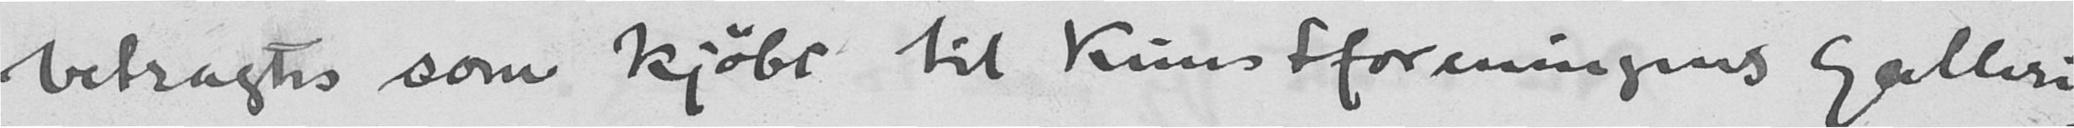betragtes som kjøbt til Kunstforeningens galleri,
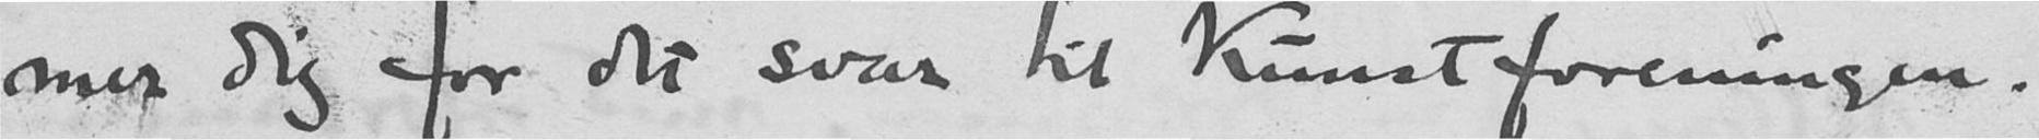mer dig for det svar til Kunstforeningen.
